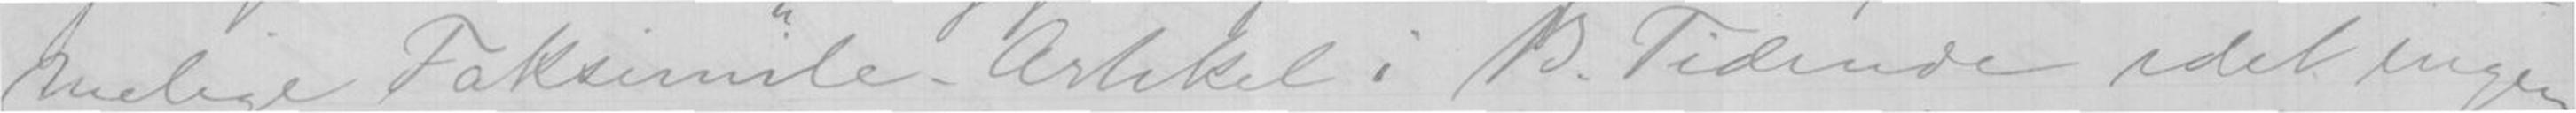melige Faksimile-Artikel i B. Tidende idet ingen
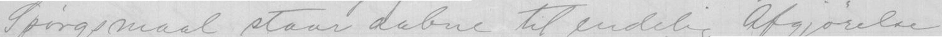Spørgsmaal staar aabne til endelig Afgjørelse
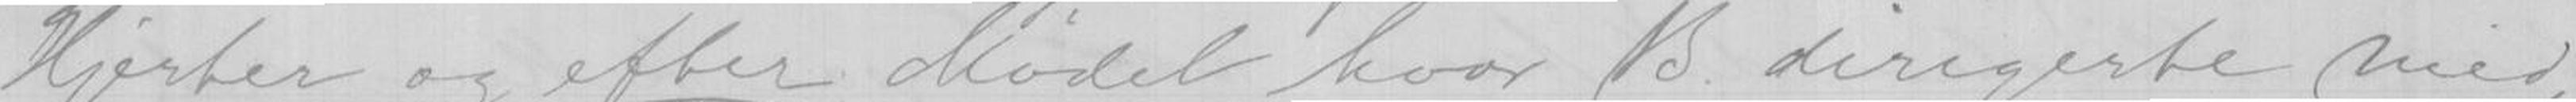Hjerter og efter Mødet, hvor B dirigerte med,
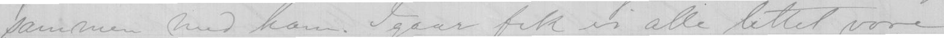sammen med ham. Igaar fik vi alle lettet vore
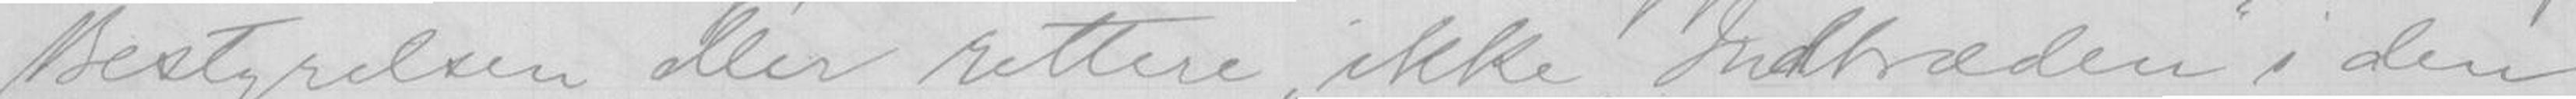Bestyrelen eller rettere ikke Indtræden i den
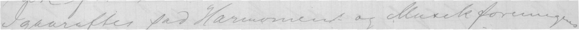Igaaraftes sad Harmoniens og Musikforeningens
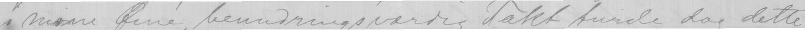i mine Øine, beundringsværdig Takt, turde dog dette
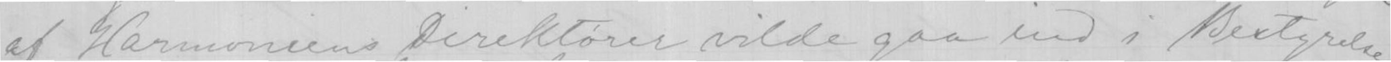af Harminiens Direktører vilde gaa ind i Bestyrelse
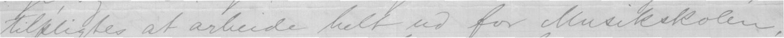tilpligtes at arbeide helt ud for Musikskolen,
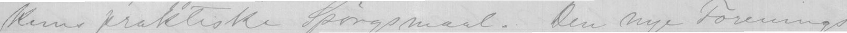kun praktiske Spørgsmaal. Den nye Forenings
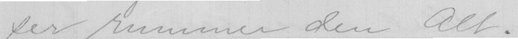ser rummer den Alt.
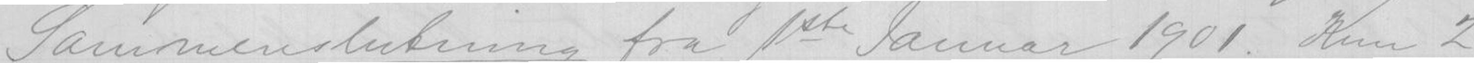Sammenslutning fra 1ste Januar 1901. Kun 2
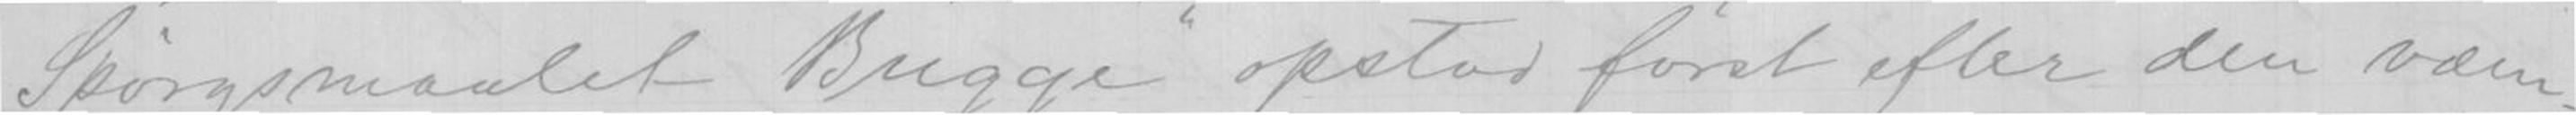Spørgsmaalet Bugge opstod først efter en væm-
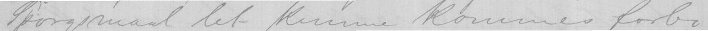Spørgsmaal let kunne kommes forbi
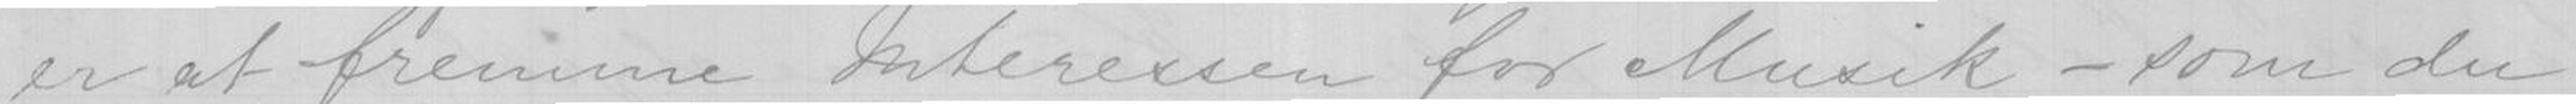er at fremme Interessen for Musik - som du
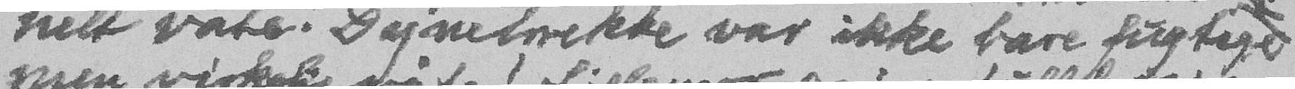helt våte! Dynetrekke var ikke bare fugtige
